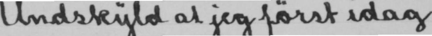Undskyld at jeg først idag
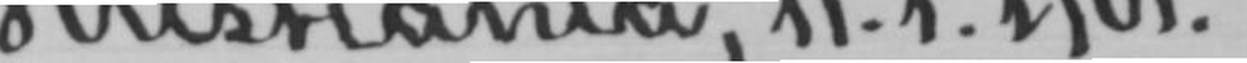Kristiania, 11.1.1901.
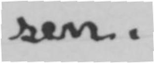sen.
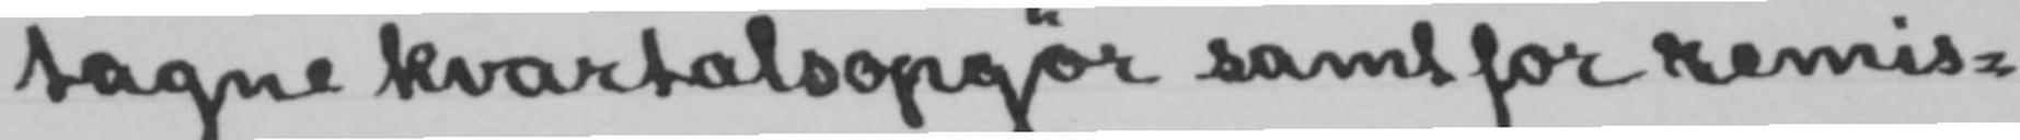tagne kvartalsopgør samt for remis-
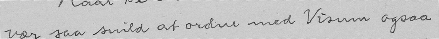vær saa snild at ordne med Visum ogsaa
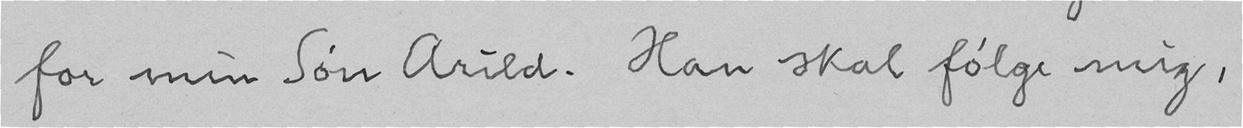for min Søn Arild. Han skal følge mig,
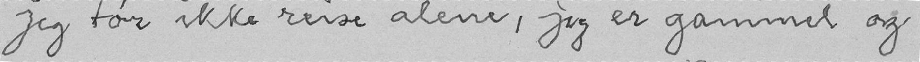jeg tør ikke reise alene, jeg er gammel og
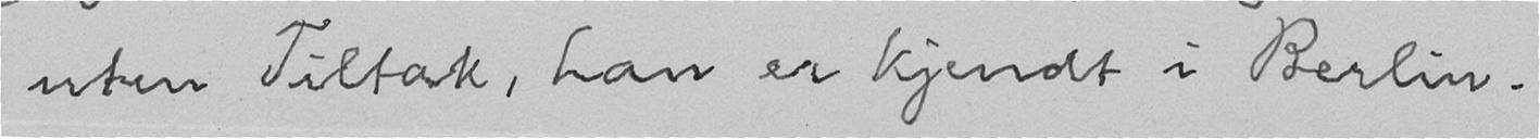uten Tiltak, han er kjendt i Berlin.
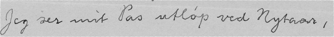Jeg ser mit Pas utløp ved Nytaar,
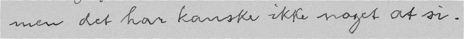men det har kanske ikke noget at si.
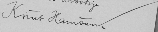Knut Hamsun.
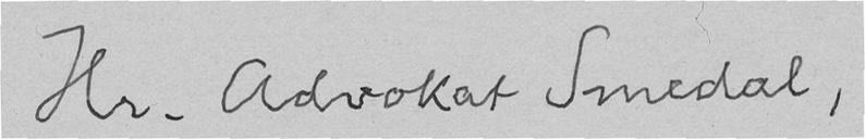Hr. Advokat Smedal,
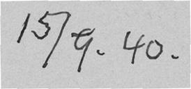15/9. 40.
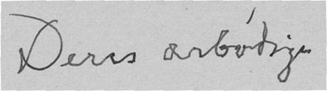Deres ærbødige
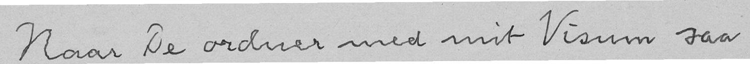Naar De ordner med mit Visum saa
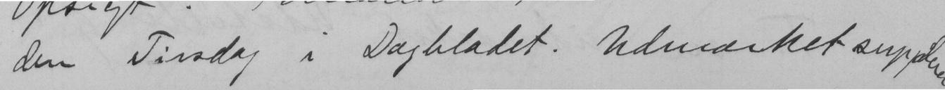den Tirsdag i Dagbladet. Udmærket supplerer
